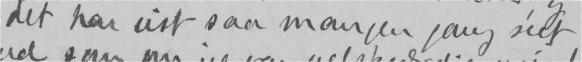det har vist saa mangen gang seet
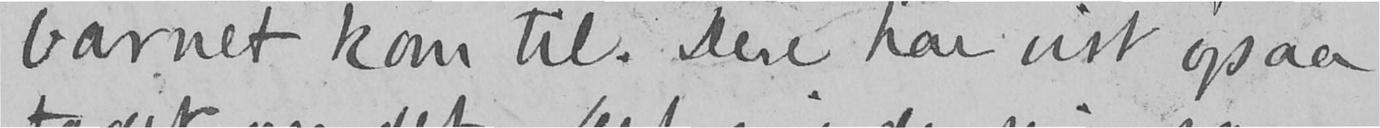barnet kom til. Dere har vist ogsaa
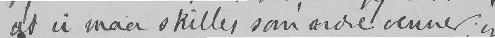at vi maa skilles som andre venner, og
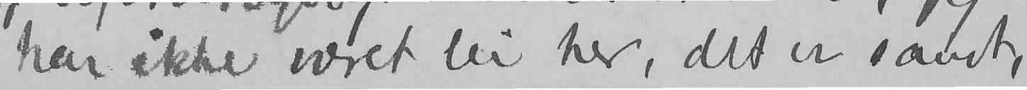har ikke været lei her, det er sandt,
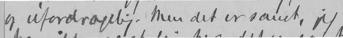og ufordragelig. Men det er sandt, jeg
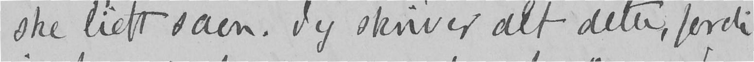ske lidt savn. Jeg skriver alt dette, fordi
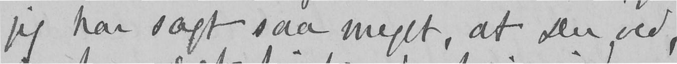jeg har sagt saa meget, at Du ved,
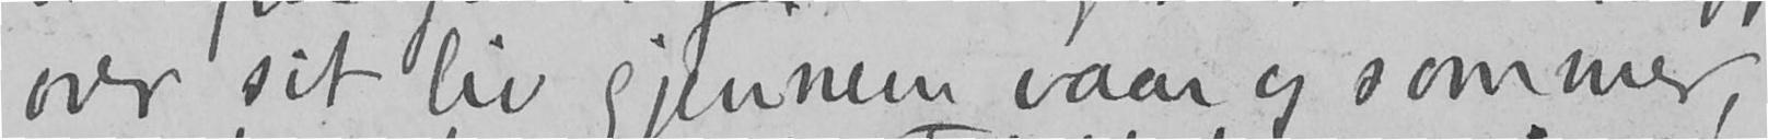over sit liv gjennem vaar og sommer,
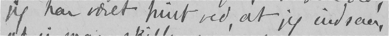jeg har været pint ved, at jeg indsaa,
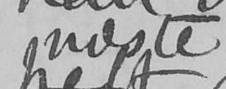næste
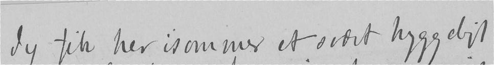Jeg fik her isommer et svært hyggeligt
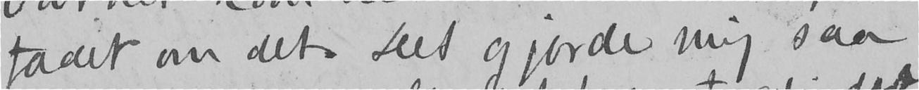faaet om det. Det gjorde mig saa
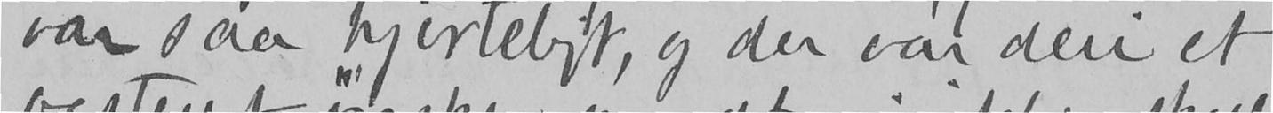var saa hjerteligt, og der var deri et
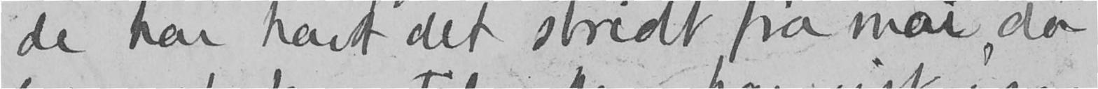de har havt det stridt fra mai, da
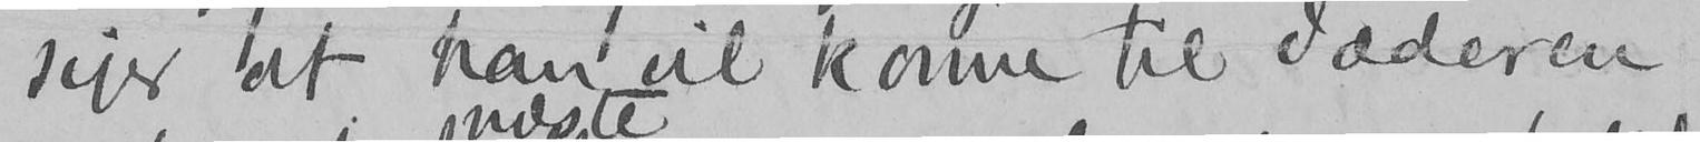siger at han vil komme til Jæderen
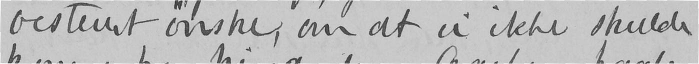bestemt ønske, om at vi ikke skulde
